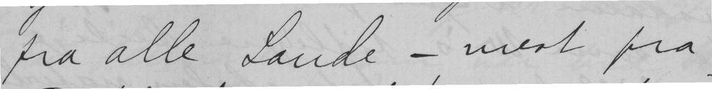fra alle Lande - mest fra
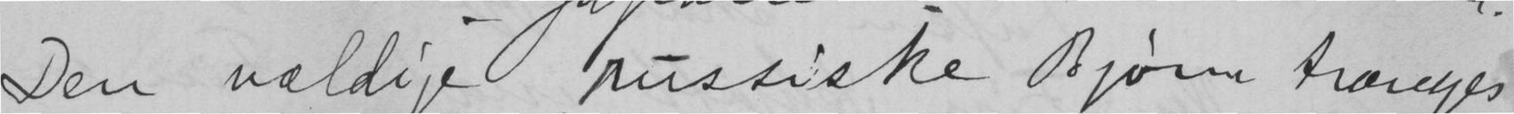Den vældige russiske Bjørn trænges
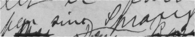for sine sprang
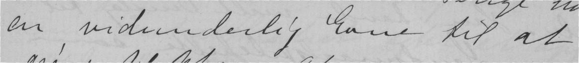en vidunderlig Evne til at
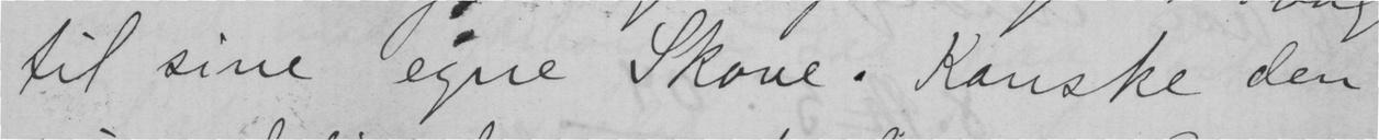til sine egne Skove. Kanske den
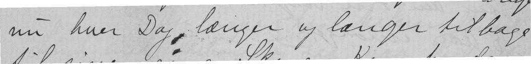nu hver Dag længer og længer tilbage
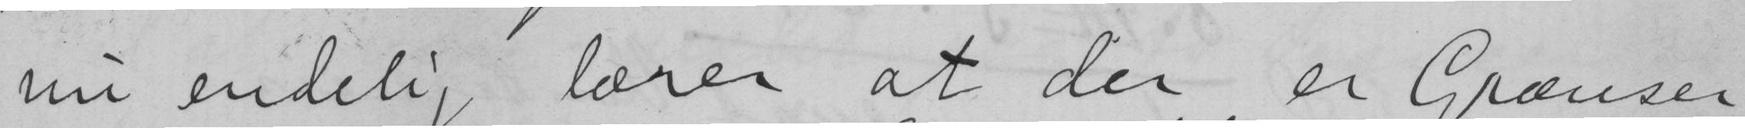nu endelig lærer at der er Grænser
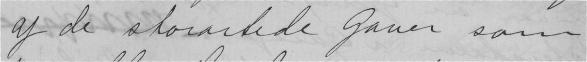af de storartede Gaver som
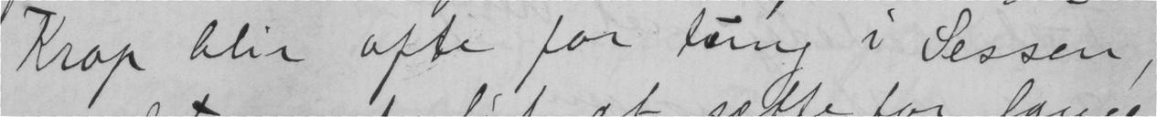Krop blir ofte for tung i Sessen,
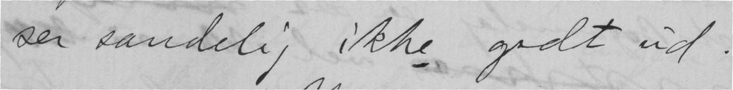ser sandelig ikke godt ud.
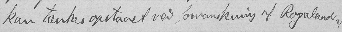kan tænkes opstaaet ved forvanskning af Rogaland?
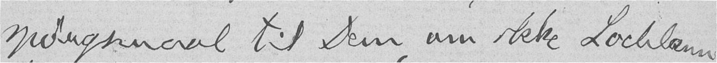spørgsmaal til Dem, om ikke Lochlann
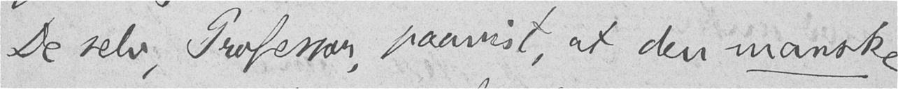De selv, Professor, paavist, at den manske
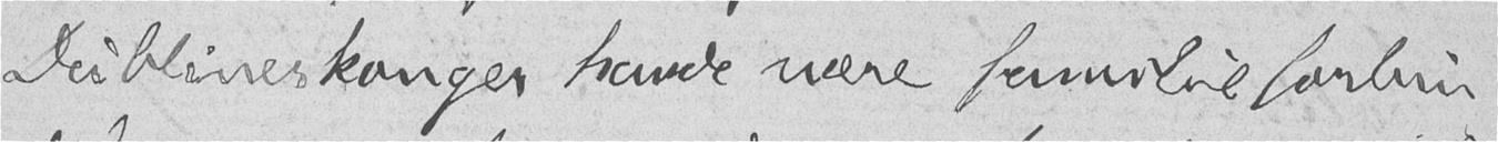Dublinerkonger havde nære familieforbin-
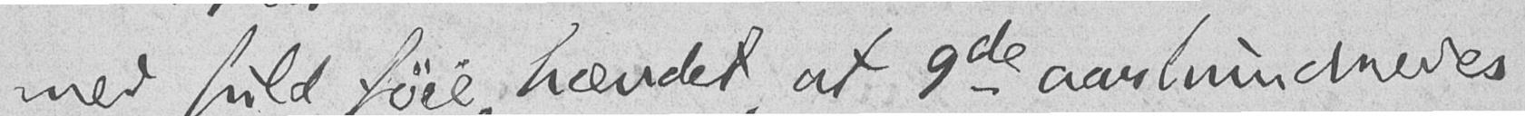med fuld føie, hævdet, at 9de aarhundredes
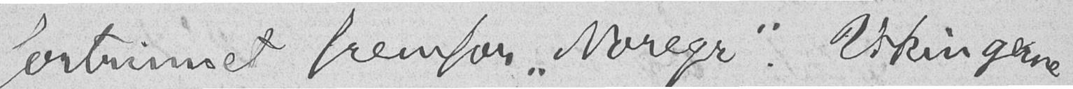fortrinnet fremfor "Noregr". Vikingerne,
